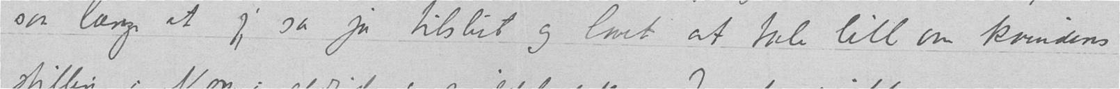saa længe at jeg sa ja tilslut og lovet at tale litt om kvindens
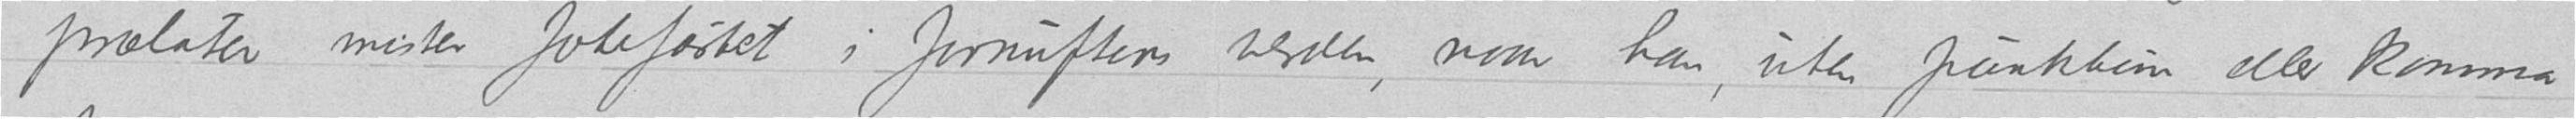prælater mister fotefæstet i fornuftens verden, naar han, uten punktum eller komma
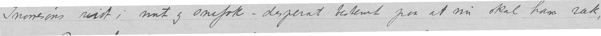Snorresons ridt i nut og snefok – desperat bestemt paa at nu skal han væk,
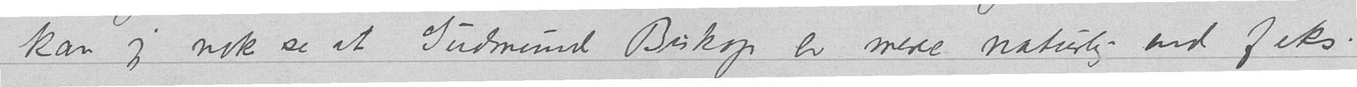kan jeg nok se at Gudmund Biskop er mere naturlig end f eks.
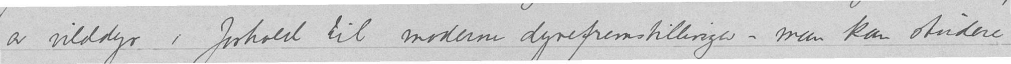av vilddyr i forhold til moderne dyrefremstillinger – man kan studere
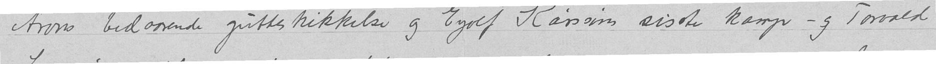Arons bedaarende gutteskikkelse og Eyolf Karssons sisste kamp – og Torvald
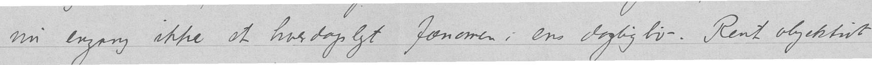nu engang ikke et hverdagsligt fænomen i ens dagligliv –. Rent objektivt
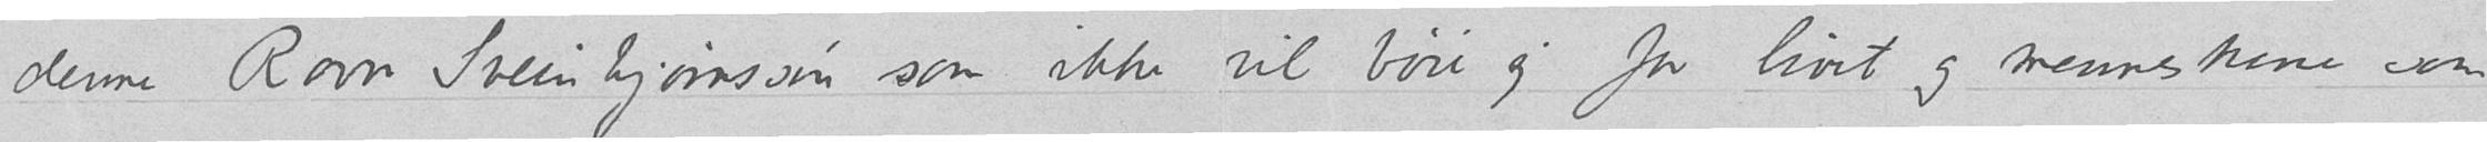denne Ravn Sveinbjørnssøn som ikke vil bøie sig for livet og menneskene som
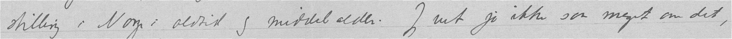stilling i Norge i oldtid og middelalder. Jeg vet jo ikke saa meget om det,
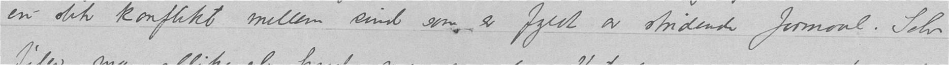en slik konflikt mellem sind som er fyldt av stridende formaal. Selv
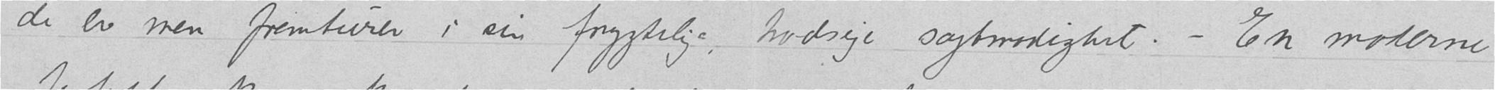de er men fremturer i sin frygtelige, trodsige sagtmodighet. – En moderne
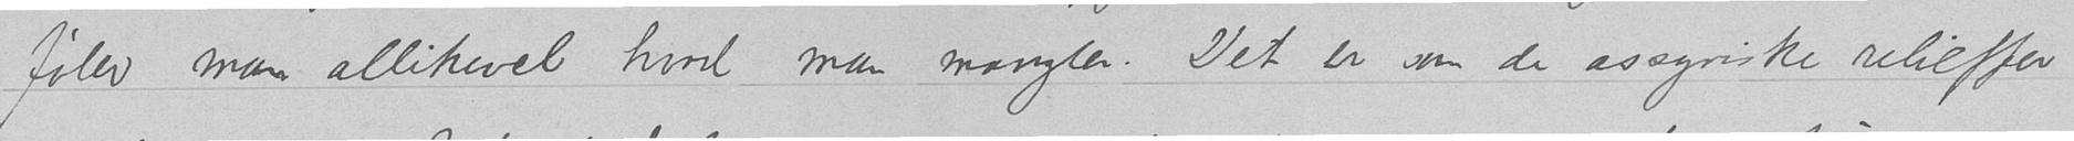føler man allikevel hvad man mangler. Det er som de assyriske relieffer
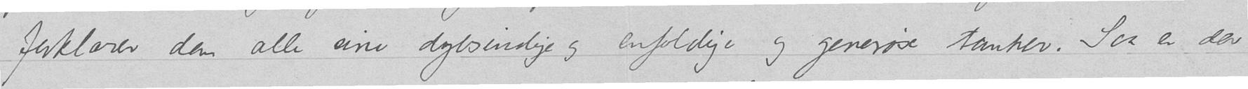forklarer dem alle sine dybsindige og enfoldige og generøse tanker. Saa er der
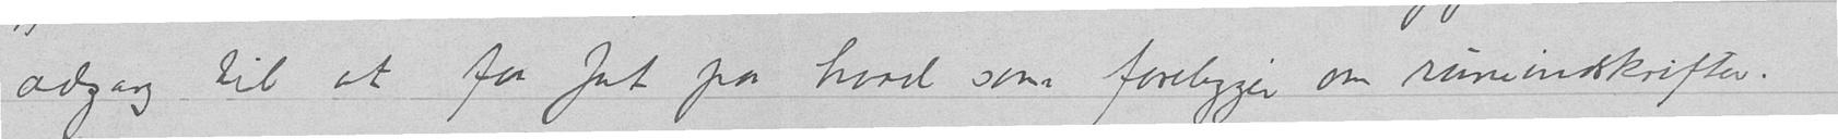adgang til at faa fat paa hvad som foreligger om runeindskrifter.
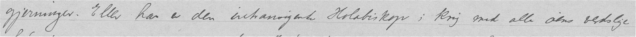gjerninger. Eller han er den intransigente Holabiskop i krig med alle øens verdslige
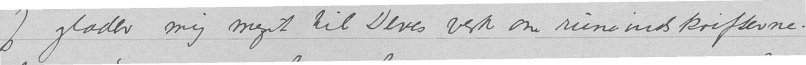Jeg glæder mig meget til Deres verk om runeindskrifterne.
